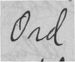Ord
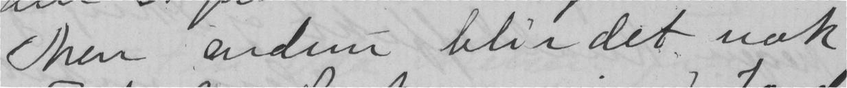Men endnu blir det nok
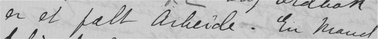er et fælt arbeide. En Mand
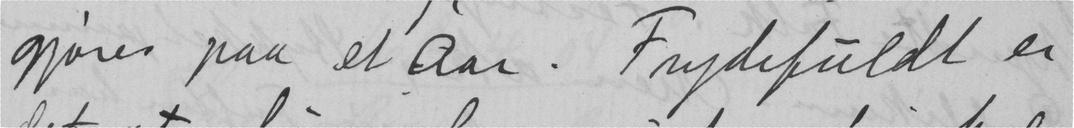gjøres paa et Aar. Frydefuldt er
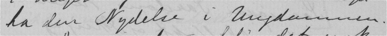ha den Nydelse i Ungdommen.
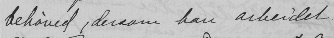behøved, dersom han arbeidet
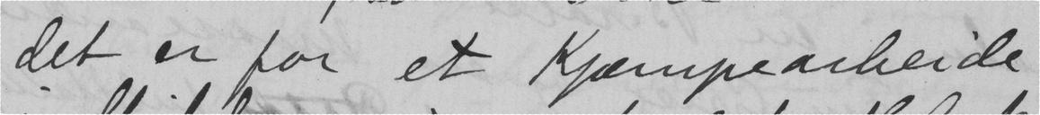det er for et Kjæmpearbeide
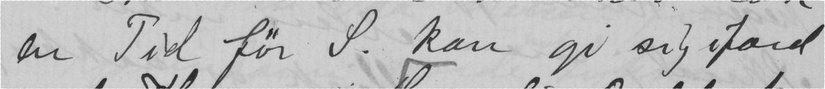en Tid før S. kan gi sig ifærd
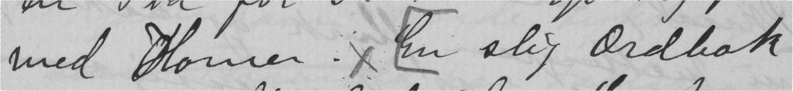med Homer. x En slig Ordbok
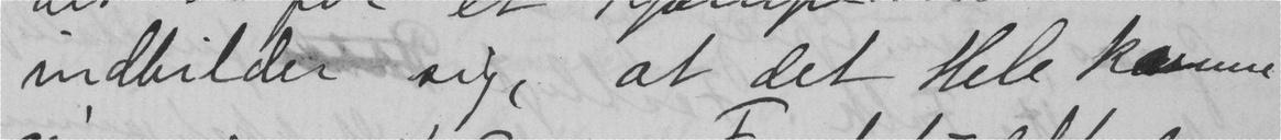indbilder sig, at det Hele kunne
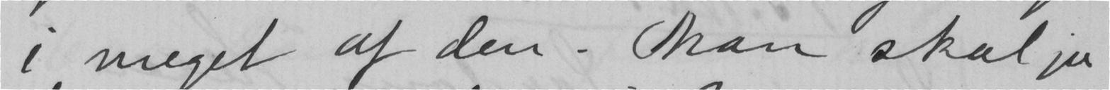i meget af den. Man skal jo
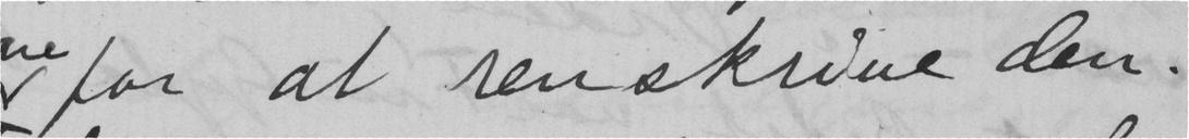for at renskrive den.
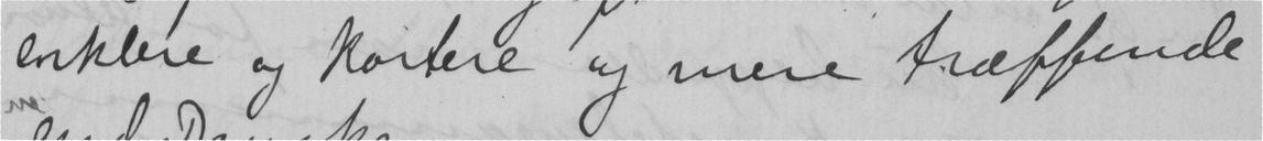enklere og kortere og mere træffende
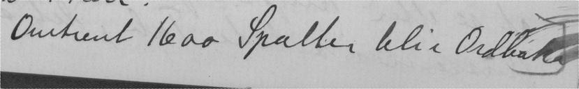Omtrent 1600 Spalter blir Ordboka
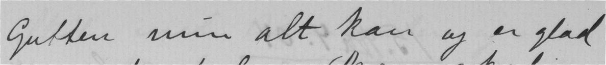Gutten min alt kan og er glad
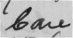bare
